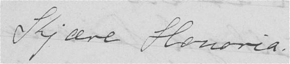Kjære Honoria!
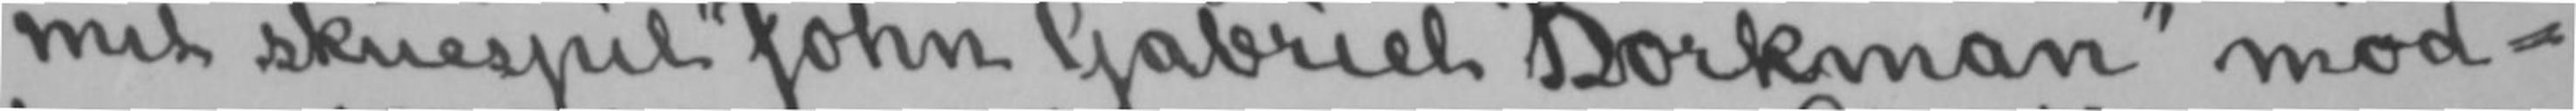mit skuespil «John Gabriel Borkman» mod-
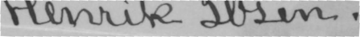Henrik Ibsen.
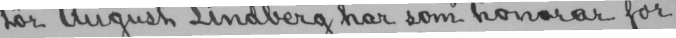tør August Lindberg har som honorar for
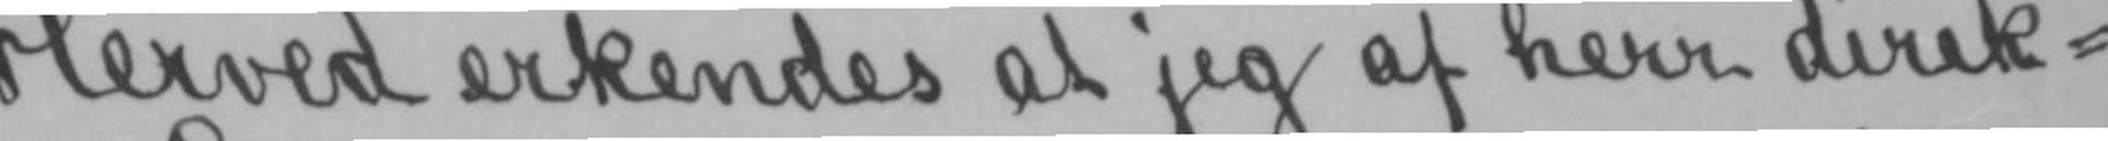Herved erkendes at jeg af herr direk-
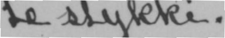te stykke.
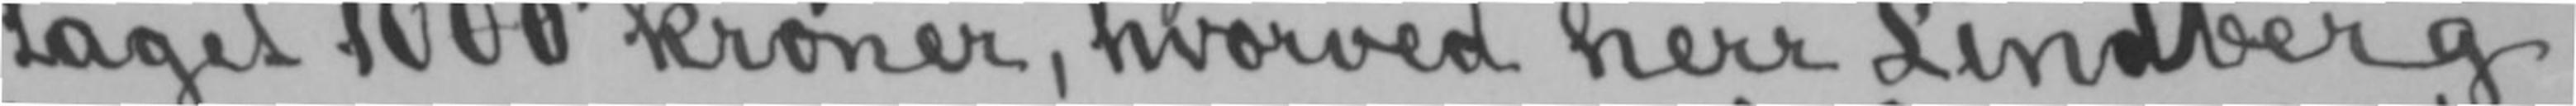taget 1000 kroner, hvorved herr Lindberg
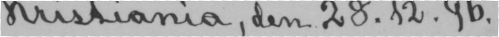Kristiania, den 28.12.96.
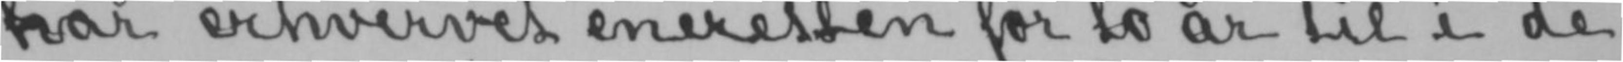har erhvervet eneretten for to år til i de
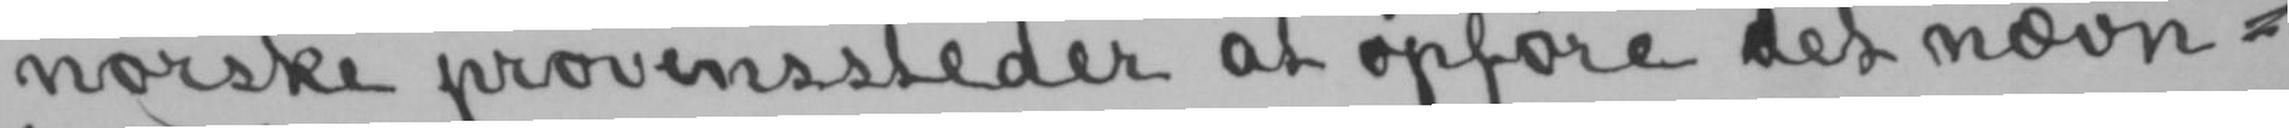norske provinssteder at opføre det nævn-
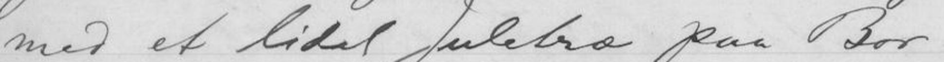med et lidet Juletræ paa Bor-
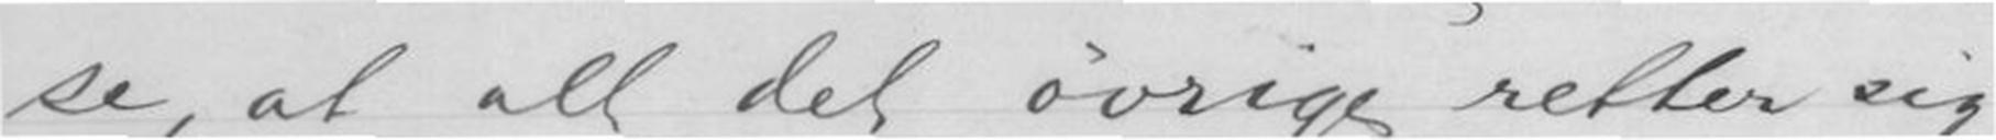se, at alt det øvrige "retter sig
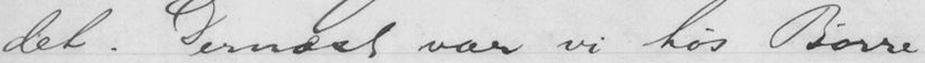det. Dernæst var vi hos Børre
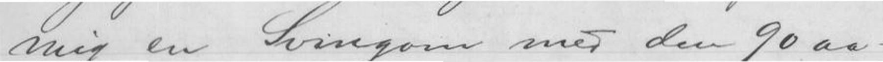mig en Svingom med den 90 aa-
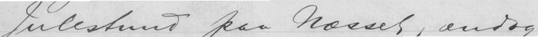Julestund paa Næsset, endog
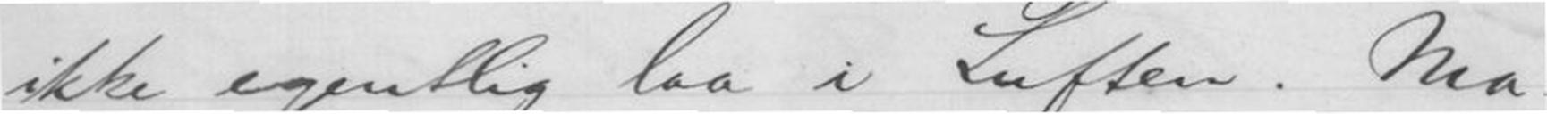ikke egentlig laa i Luften. Ma-
